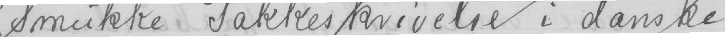Smukke Takkeskrivelse i danske
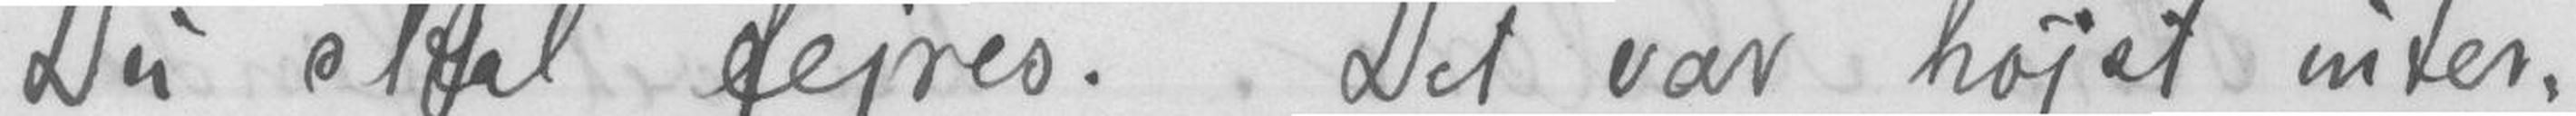Du skal feires. Det var højst inter-
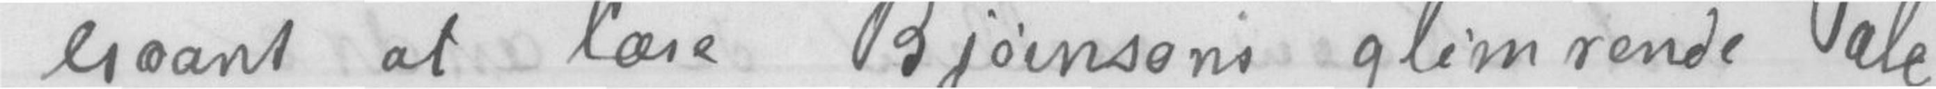essant at lære Bjørnsons glimrende Tale,
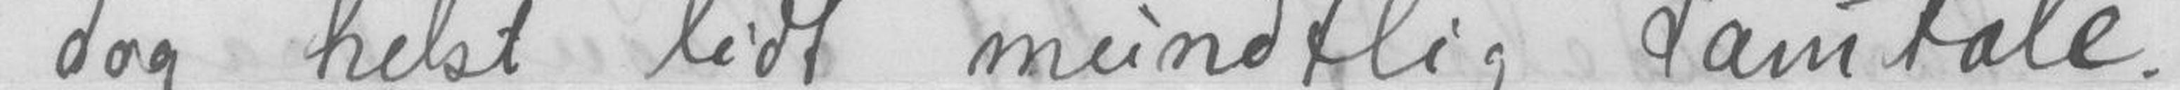dog helst lidt mundtlig Samtale.
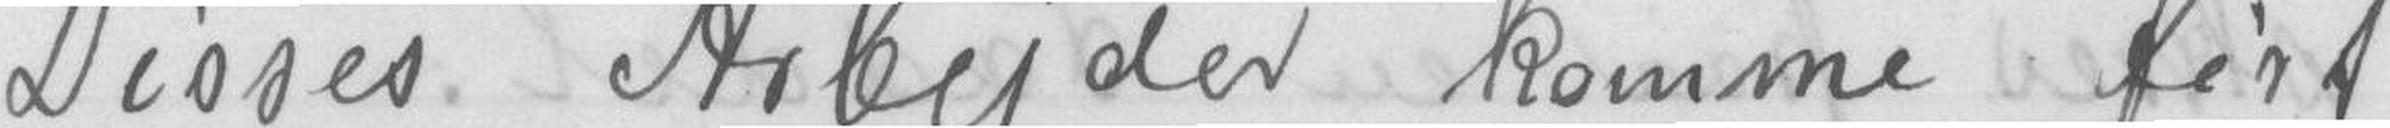Disses Arbejder kommer fint
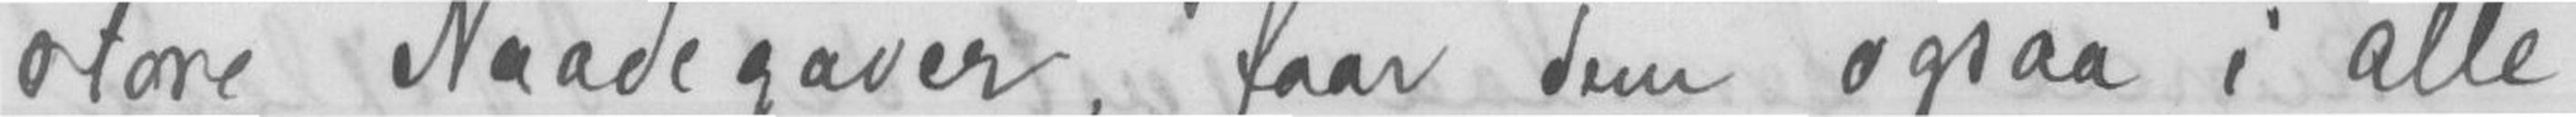store Naadegaver, faar den ogsaa i alle
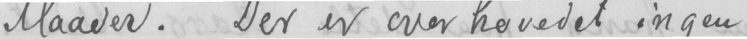Maader. Der er overhovedet ingen
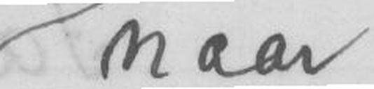naar
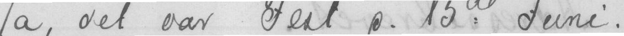Ja, det var Fest p.15de Juni.
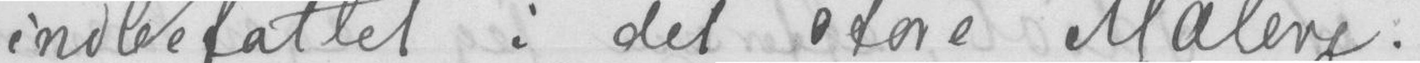indbefattet i det store Maleri.
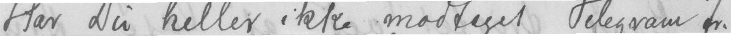Har Du heller ikke modtaget Telegram fra
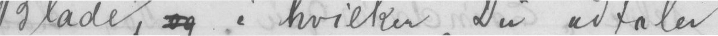Blade og i hvilken Du udtaler
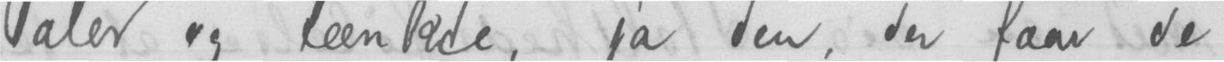Taler og tænkte, ja den, der faar de
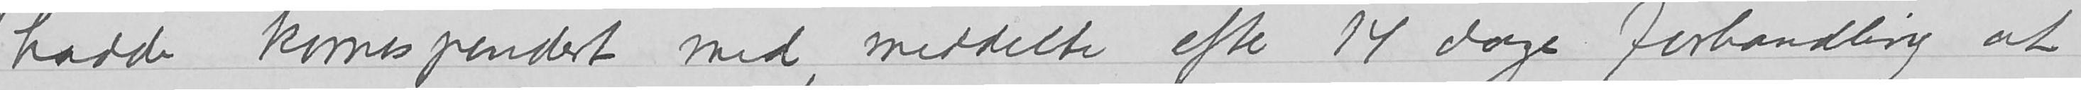hadde korrespondert med, meddelte efter 14 dagesr forhandling at
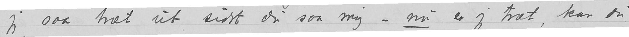jeg saa træt ut sidst du saa mig – nu er jeg træt, kan du
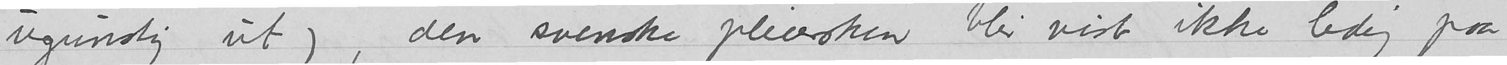ugunstig ut), den svenske pleiersken blir vist ikke ledig paa
